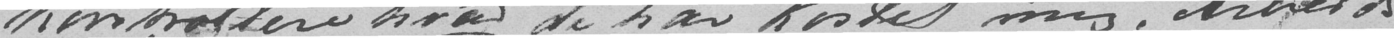kontrollere hvad de har kostet mig, Arbeid og
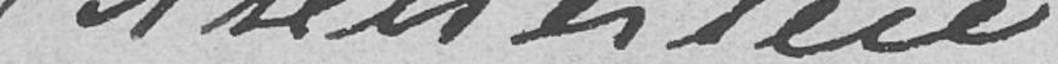Atelierleie
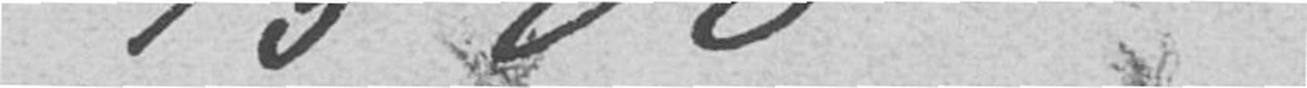1500
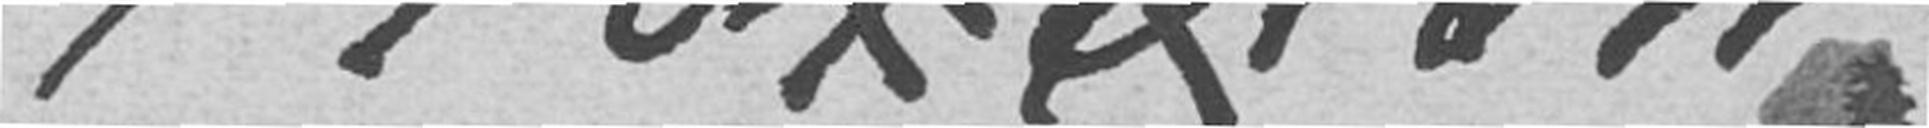110461 Kr
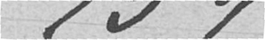157
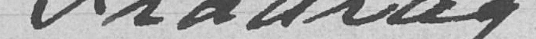Fradrag
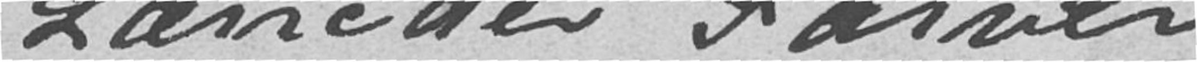Lærreder Farver
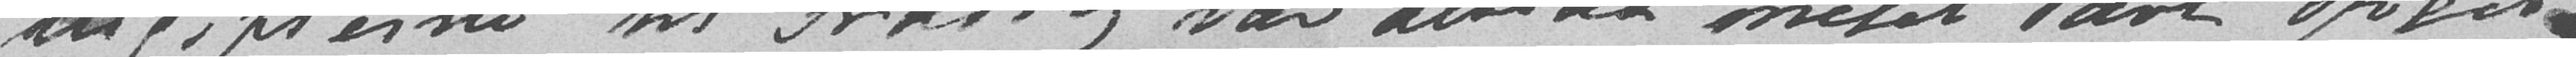udgifterne til Fradrag var altsaa meget lavt opgit
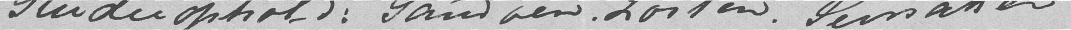Studieophold: Sandven. Løiten. Jevnaker
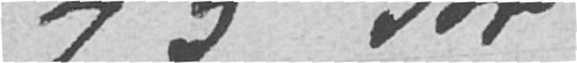45 kr
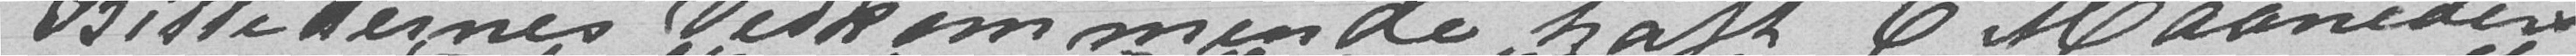Billedernes Vedkommende haft 6 Maanders
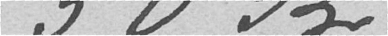50 Kr
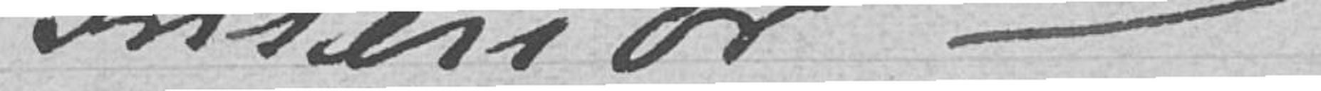Interiør
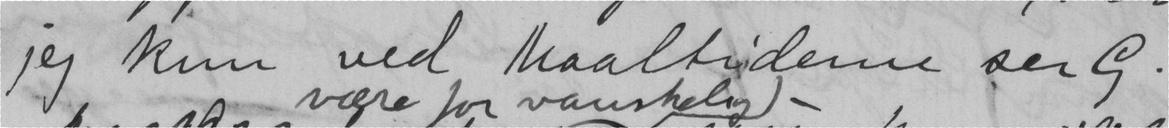jeg kun ved maaltiderne ser G.
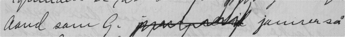Aand som G.  jamrer så
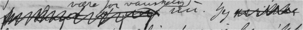nu. Jeg
